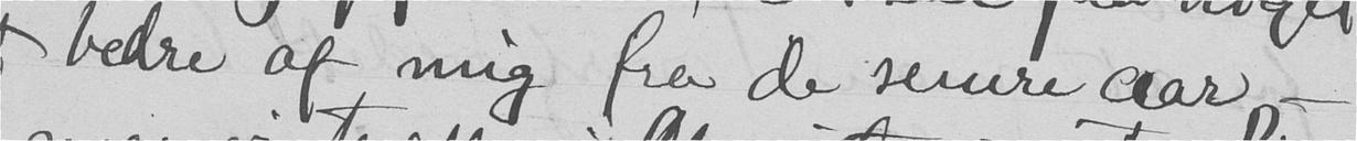bedre af mig fra de senere aar -
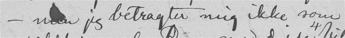- men jeg betragter mig ikke som
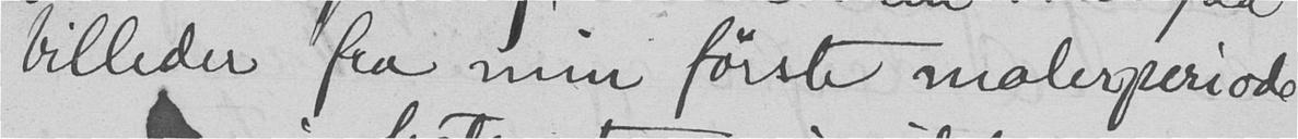billeder fra min første malerperiode
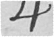4
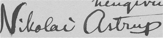Nikolai Astrup
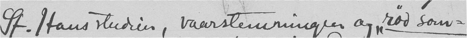St. Hans studien, vaarstemningen og "rød som-
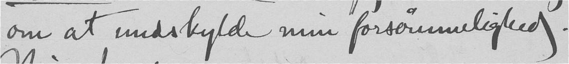om at undskylde min forsømmelighed.
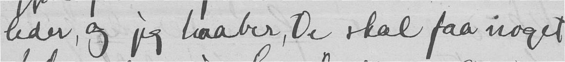leder, og jeg haaber, De skal faa noget
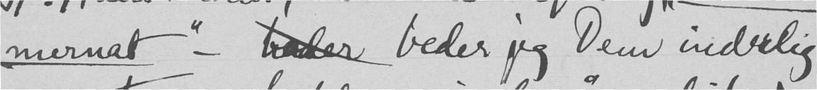mernat" -  beder jeg Dem inderlig
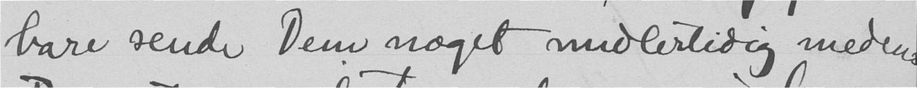bare sende Dem noget midlertidig medens
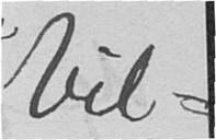bil-
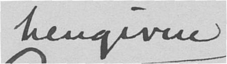hengivne
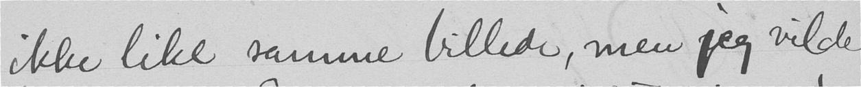ikke like samme billede, men jeg vilde
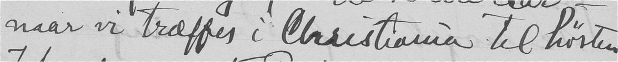naar vi træffes i Christiania til høsten
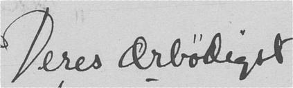Deres ærbødigst
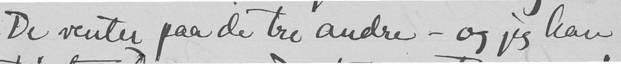De venter paa de tre andre - og jeg kan
